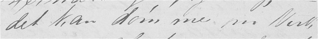det kan dømme om Virk-
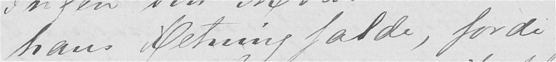hans Retningfalde, fordi
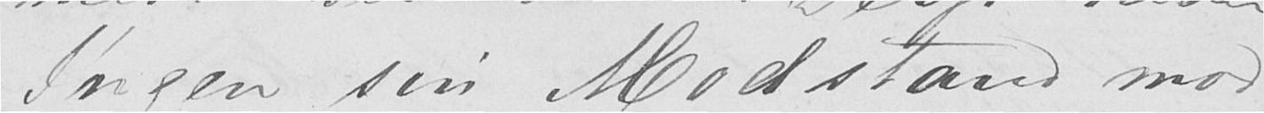Ingen sin Modstand mod
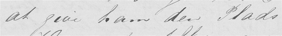at give ham den Plads
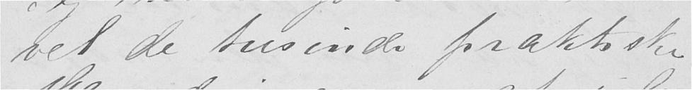vel de tusende praktiske
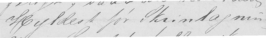Hyldest før Skrinlagning
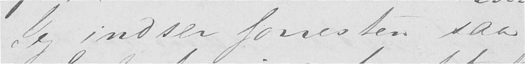Jeg indser forresten saa
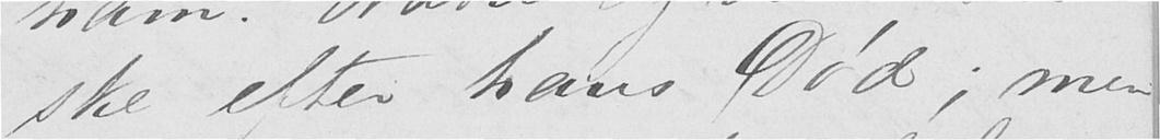ske efter hans Død; men
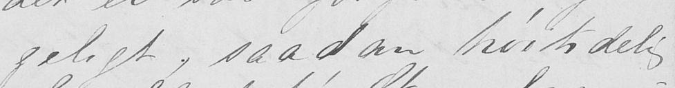geligt, saadan høitidelig
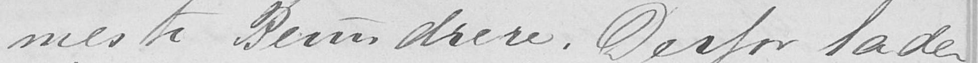meste Beundrere. Derfor lader
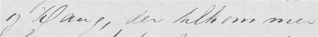og Rang, der tilkommer
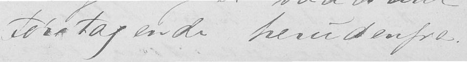Foretagende herudenfra.
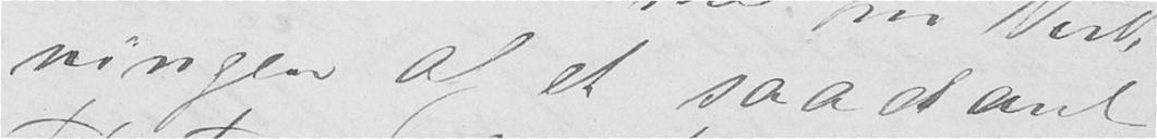ningen af et saadant
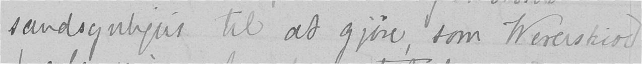sandsynligvis til at gjøre som Werenskiold
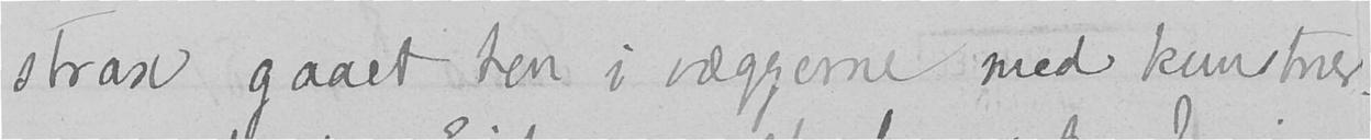strax gaaet hen i væggerne med kunstner-
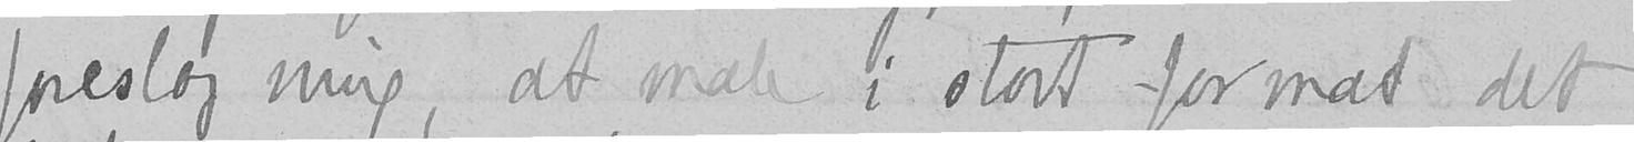foreslog mig, at male i stort format det
